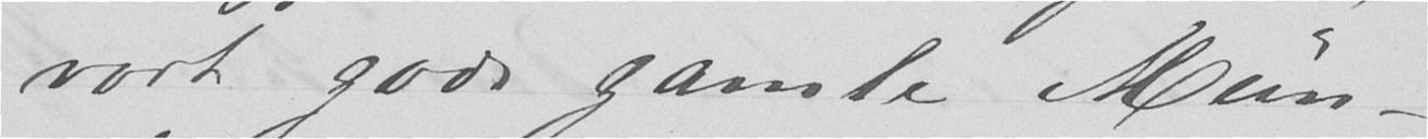vort gode gamle Mün-
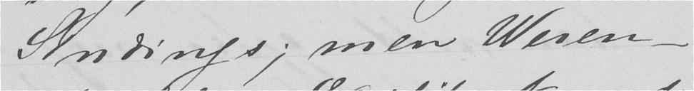Sindings; men Weren-
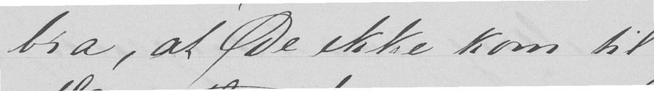bra, at De ikke kom til
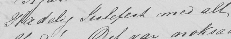Glædelig Julefest med alt
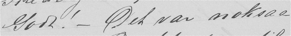Godt! - Det var noksaa
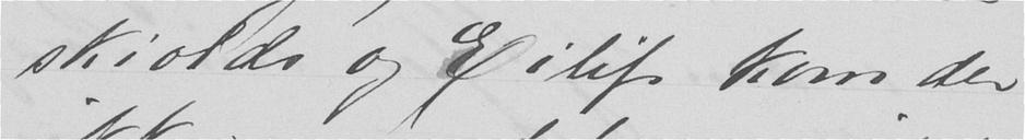skiolds og Eilif kom der
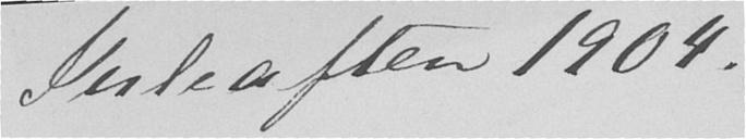Juleaften 1904.
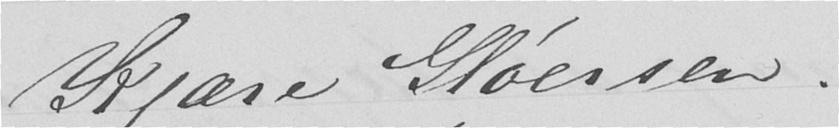Kjære Gløersen.
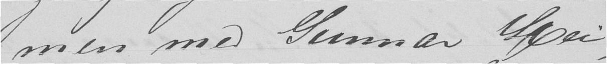men med Gunnar Hei-
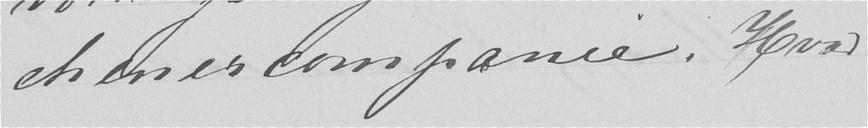chenescompanie. Hvad
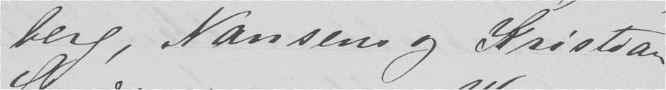berg, Nansens og Kristian
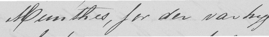Munthes, for der var hyg-
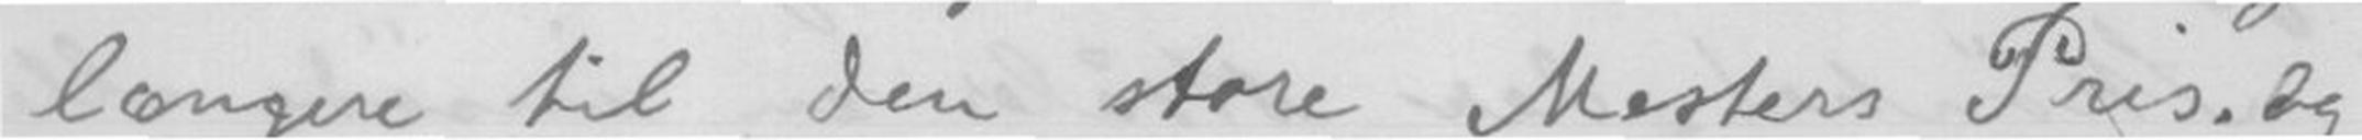længere til den store Mester Pris. Dog
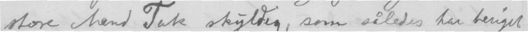store Mand Tak skyldig, som således har beriget
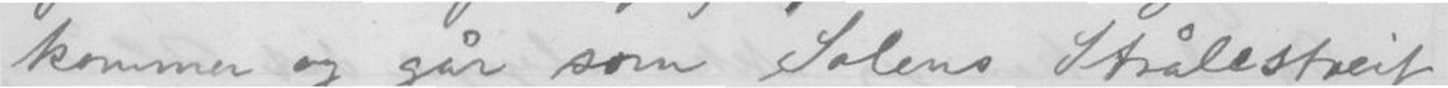kommer og går som Solens Strålestreif
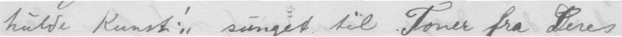hulde Kunst!" sunget til Toner fra Deres
